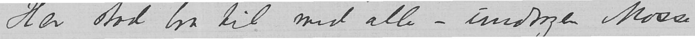Her stod bra til med alle – undtagen Mosse,
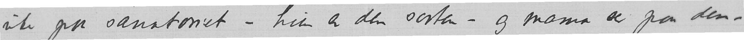ute paa sanatoriet – hun er den sorten – og mama ser paa dem –
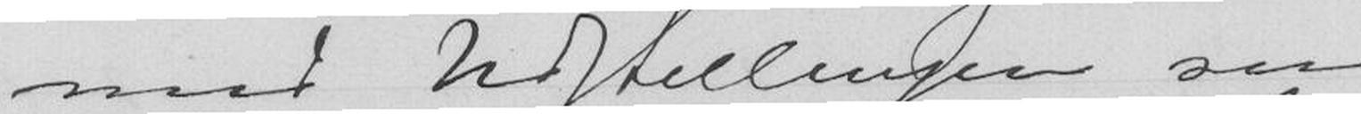med Udstillingen som
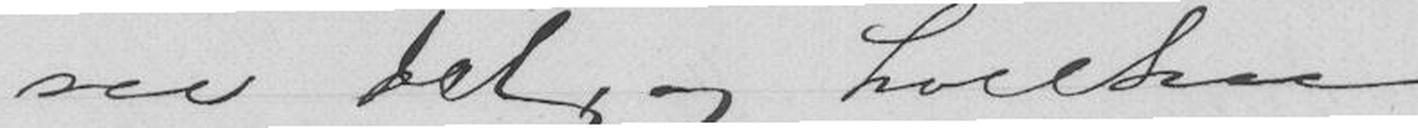see det, og hvilken
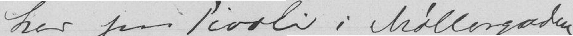her paa Tivoli i Møllergaden
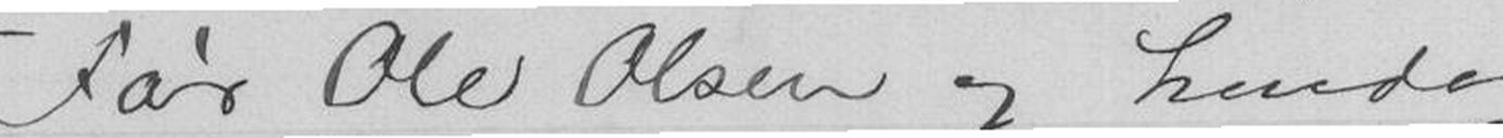Fàr Ole Olsen og hendes
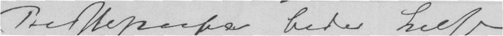Bestepapa beder hilse
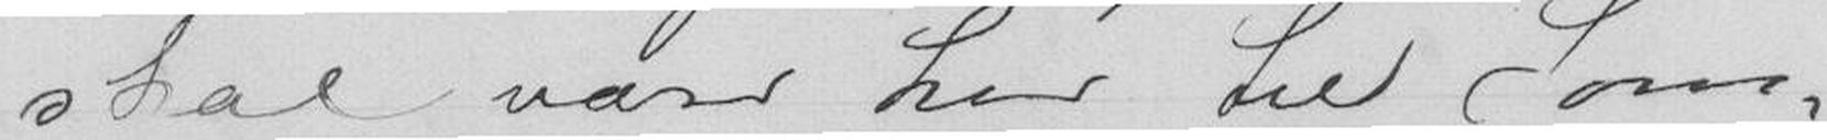skal være her til Som-
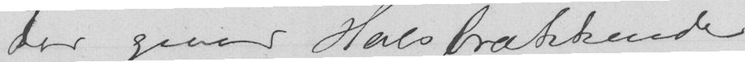der giver Halsbrækkende
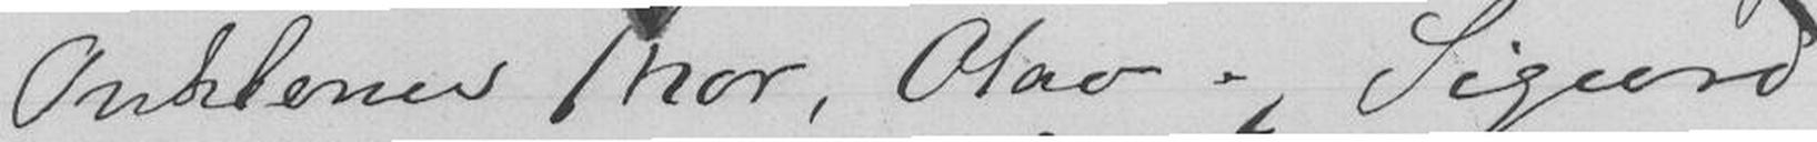Onklener Thor, Olav og Sigurd
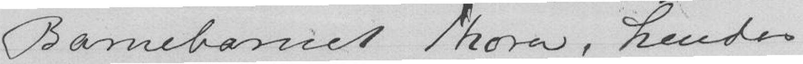Barnebarnet Thora, hendes
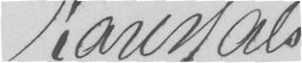Karl Hals
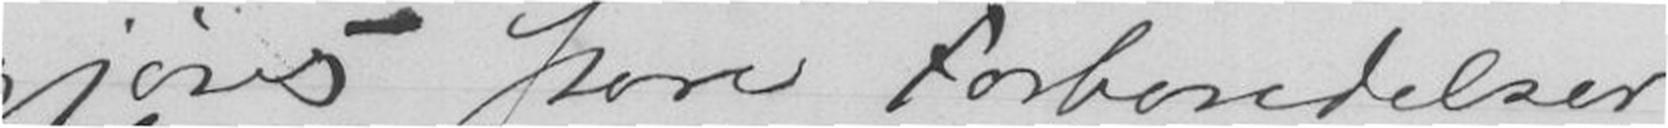gjøres store Forbindelser
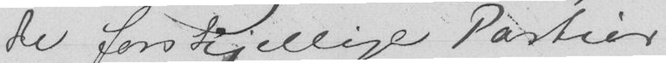De forskjellige Partier
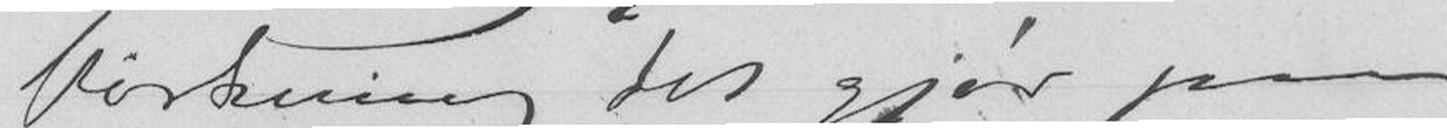Virkning det gjør paa
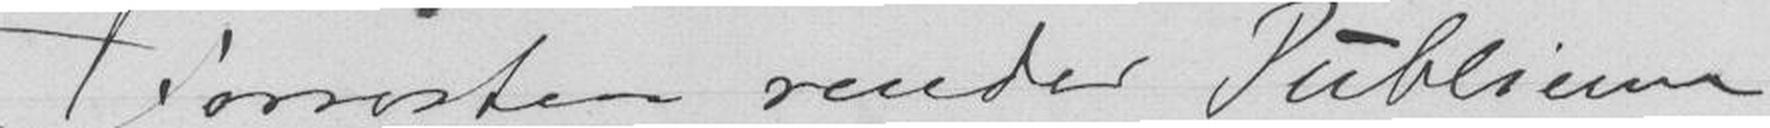Forresten sender Publicun
